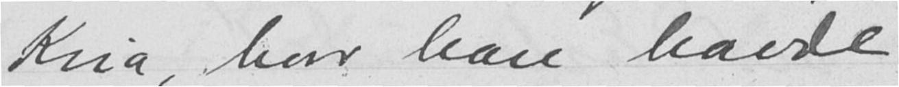Kria, hvor han havde
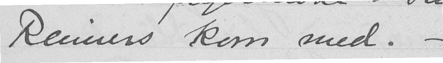Reimers kom med. -
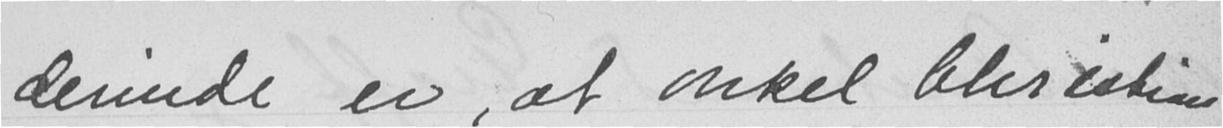derinde er, at onkel Christian
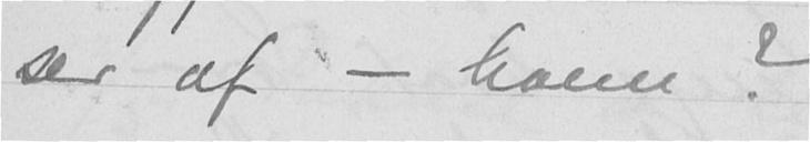ser af - ham?
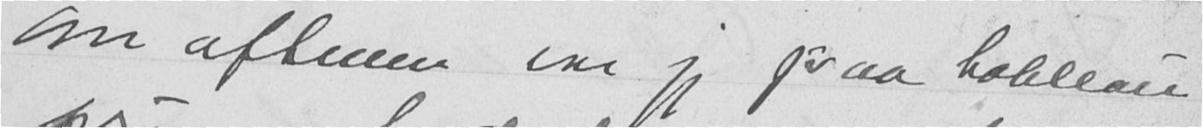Om aftenen var jeg paa tableau
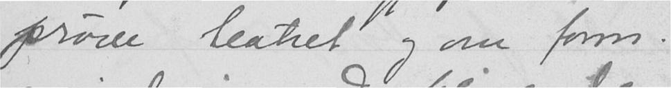prøve teatret og om form.
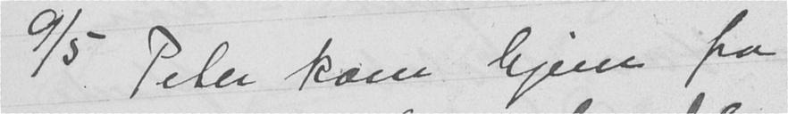9/5 Peter kom hjem fra
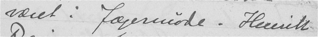været i Jægermøde. Henrik
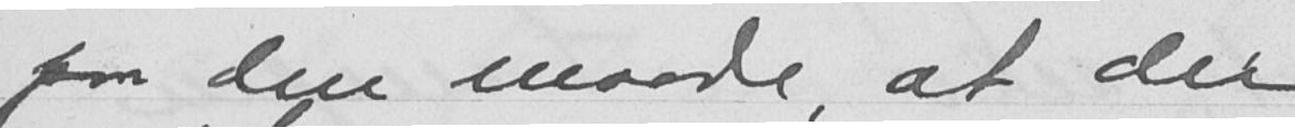paa den maade, at der
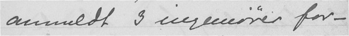anmeldt 3 ingeniører for -
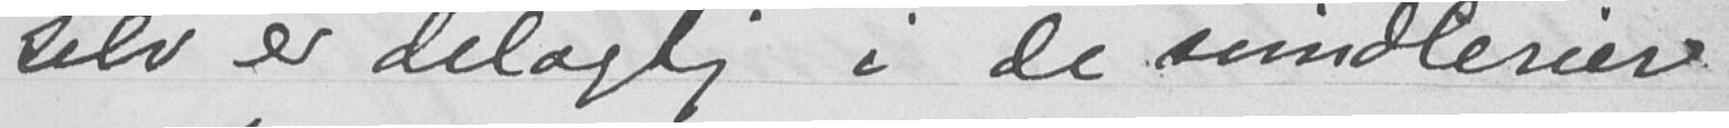selv er delagtig i de svindlerier
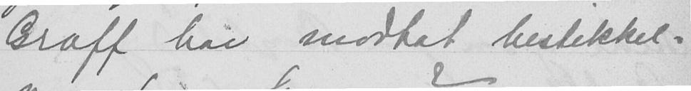Graff har modtat bestikkel-
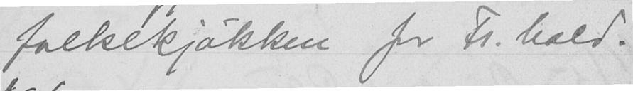folkekjøkken for Fr. hald.
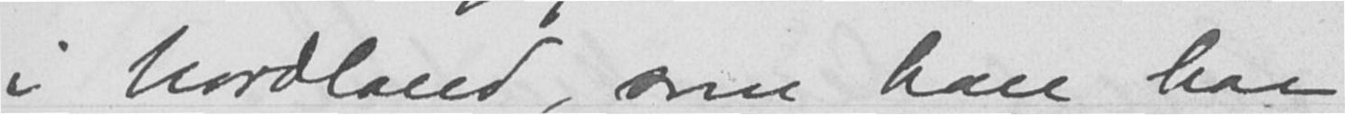i Nordland, som han har
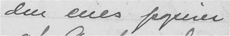den enes papirer
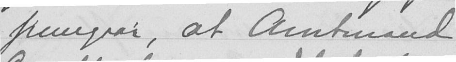fremgaar, at Amtmand
